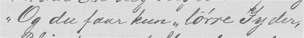"Og du faar kun "tørre Jyder,
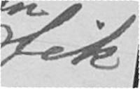fik
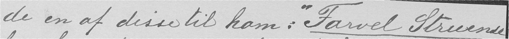de en af disse til ham: "Farvel Struense"
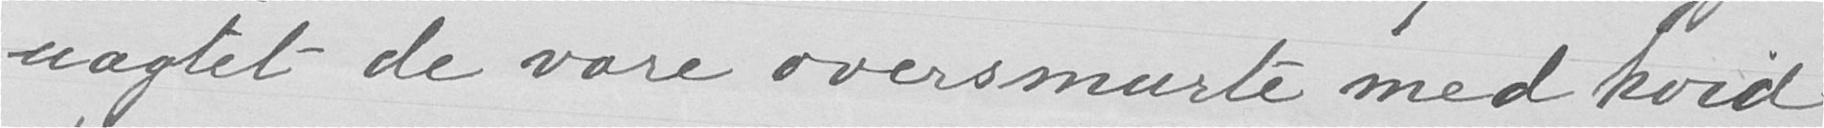uagtet de vare oversmurte med hvid
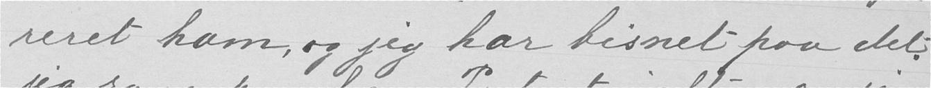reret ham, og jeg har bisnet paa det
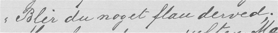"Blir du noget flau derved.
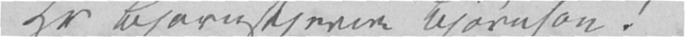Hr Bjørnstjerne Bjørnson!
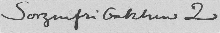Sorgenfribakken 2
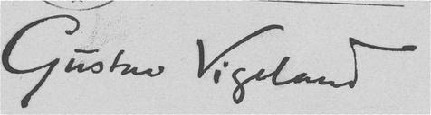Gustav Vigeland
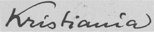Kristiania
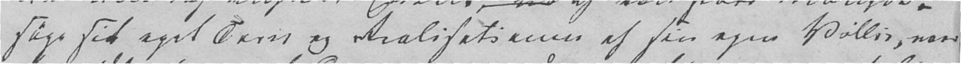søge sit eget Tarv og Realisationen af sin egen Villie, naar
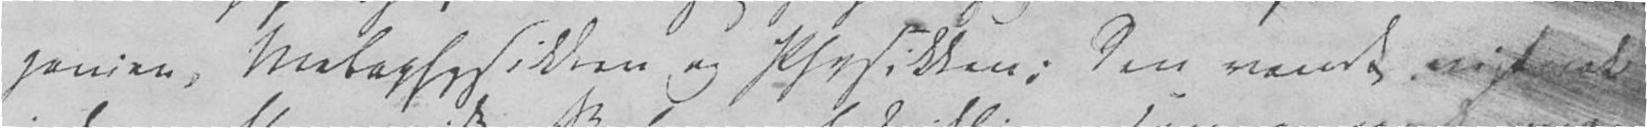gonien, Metaphysikken og Physikken: Den vandt vistnok
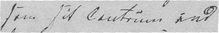som sit Centrum end
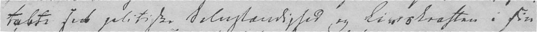tabte sin politiske Selvstændighed og Livskraften i sin
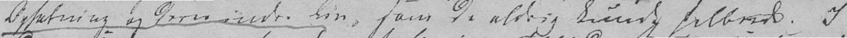Opfatning og deres indre Liv, som de aldrig kunde helbrede. I
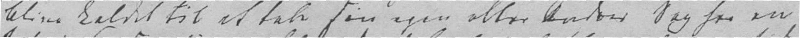blive kaldet til at tale sin egen eller Andres Sag for en
